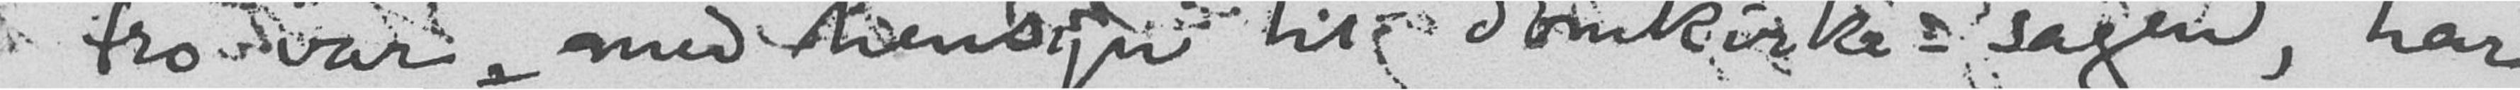tro var, med hensyn til domkirke-sagen, har
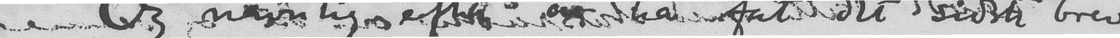Og nævnlig efter at ha fåt dit sidste brev
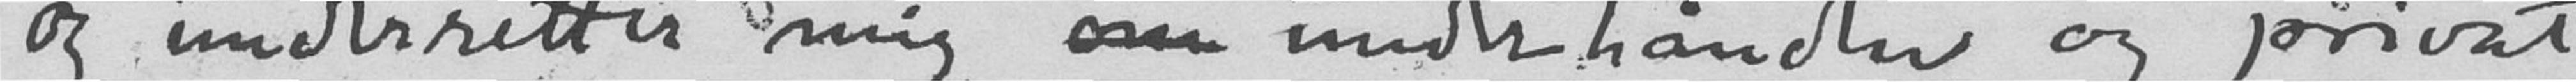og underretter mig om underhånden og privat
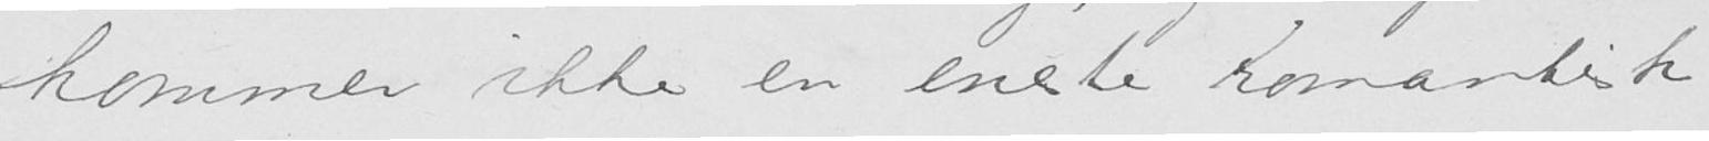kommer ikke en eneste romantisk
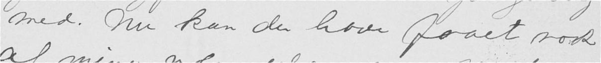med. Nu kan du have faaet nok
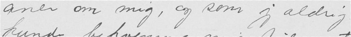aner om mig, og som jeg aldrig
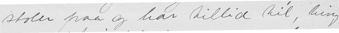stoler paa og har tillid til, ting
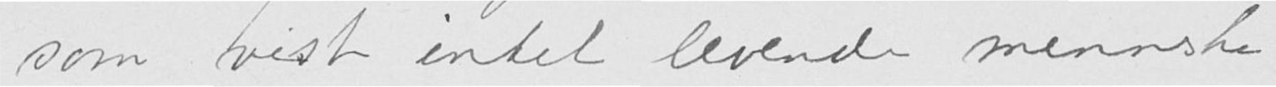som vist intet levende menneske
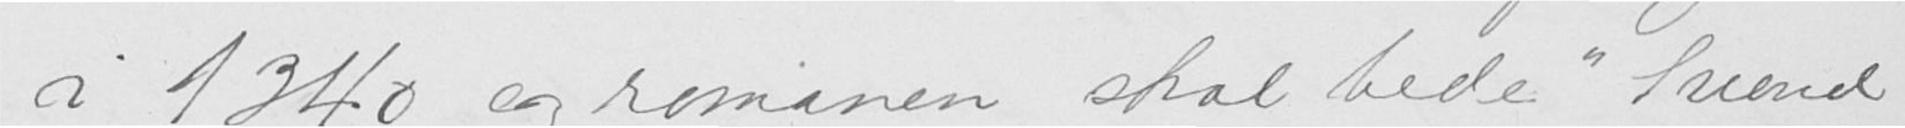i 1340 og romanen skal hede "Svend
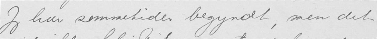Jeg har sommetider begyndt, men det
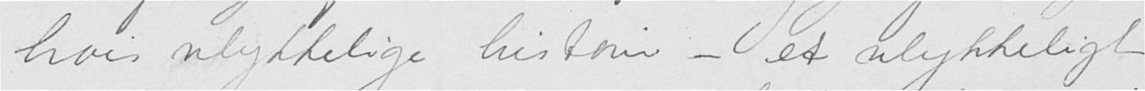hvis ulykkelige historie - et ulykkeligt
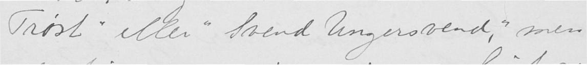Trøst" eller Svend Ungersvend," men
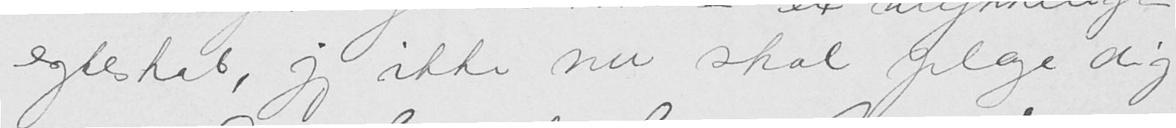egteskab, jeg ikke nu skal plage dig
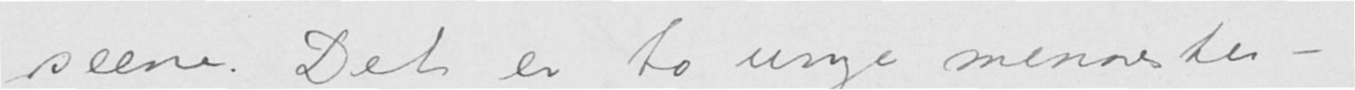scene. Det er to unge mennesker -
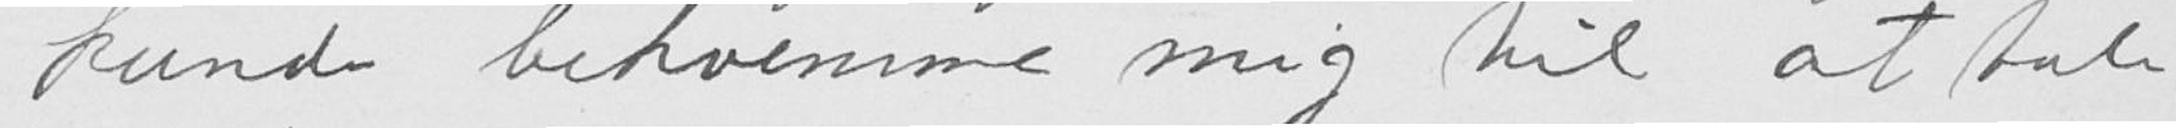kunde bekvemme mig til at tale
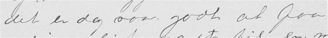det er dog saa godt at faa
